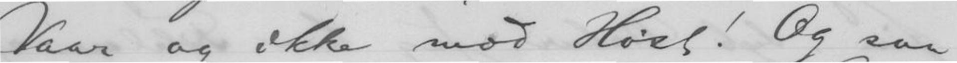Vaar og ikke mod Høst! Og saa
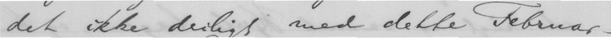det ikke deiligt med dette Februar-
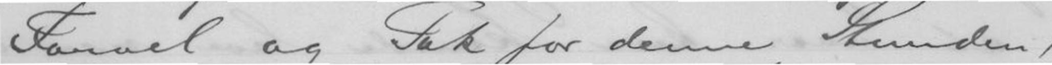Farvel og Tak for denne Stunden! Marie
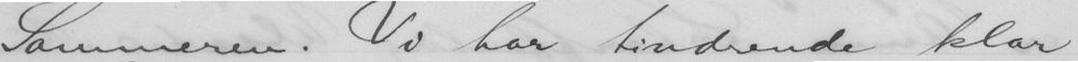Sommeren. Vi har tindrende klar
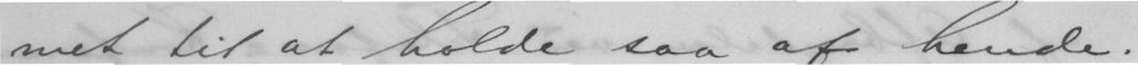met til at holde saa af hende.
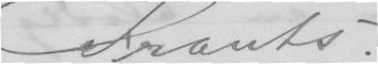Frants.
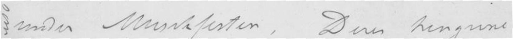i under Musikfesten. Deres hengivne
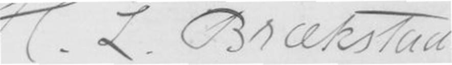H.L. Brækstad
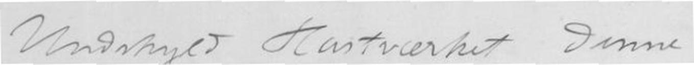Undskyld Hastværket Denne
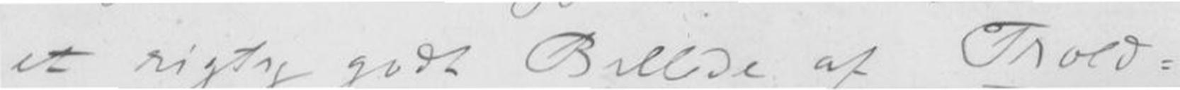et rigtig godt Billede af Trold-
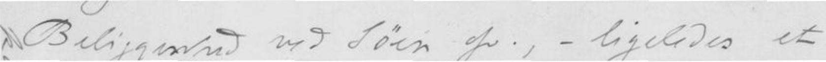Beliggenhed ved Søen osv., - ligeledes et
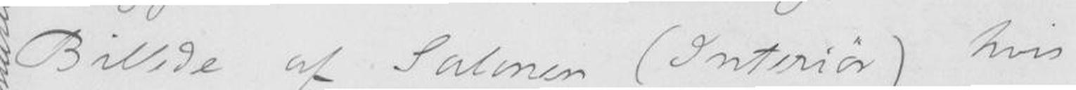Billede af Salmen (Interiør) hvis
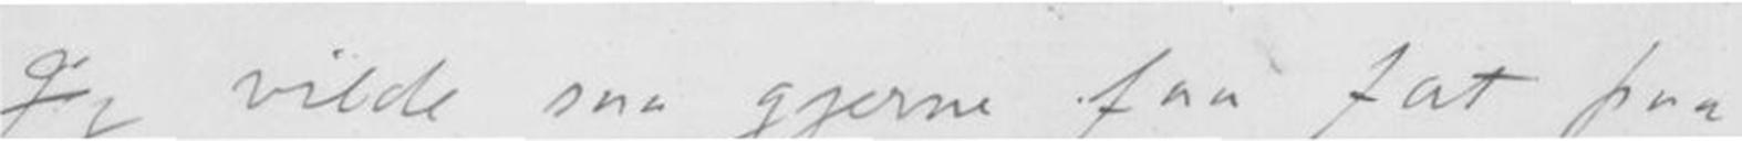Jeg vilde saa gjerne faa fat paa
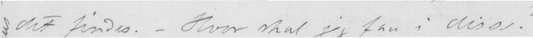det findes. - Hvor skal jeg faa i disse?
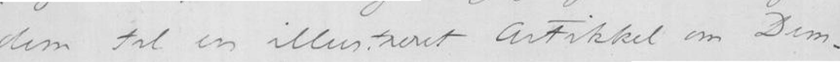dem til en illustreret Artikkel om Dem.
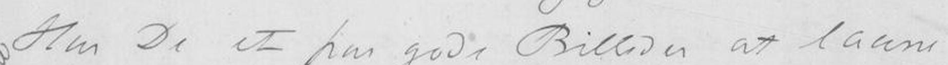Har De et par gode Billeder at laane
